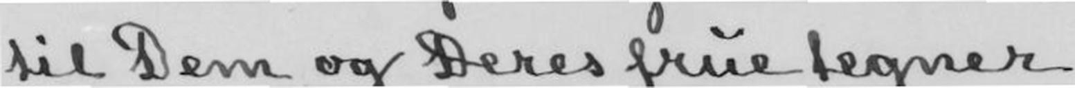til Dem og Deres frue tegner
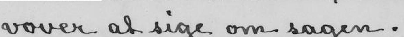vover at sige om sagen.
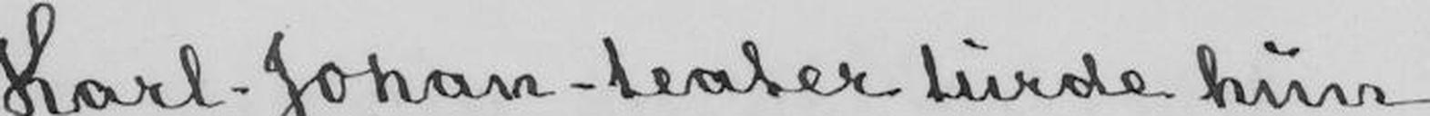Karl-Johan-teater turde hun
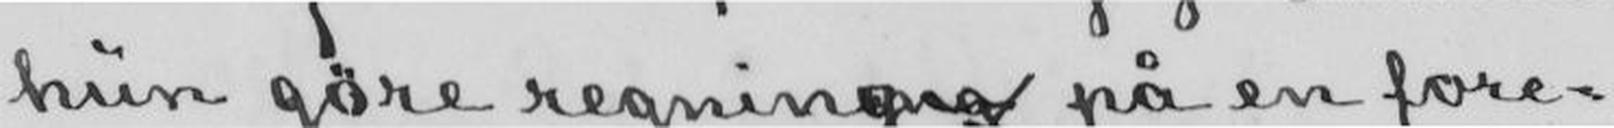hun gøre regningng på en fore-
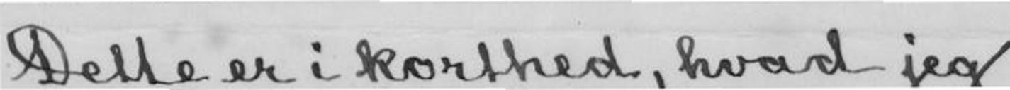Dette er i korthed, hvad jeg
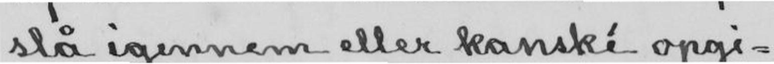slå igennem eller kanské opgi-
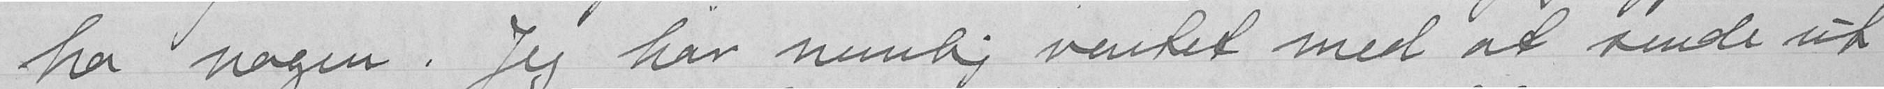fra nogen. Jeg har nemlig ventet med at sende ut
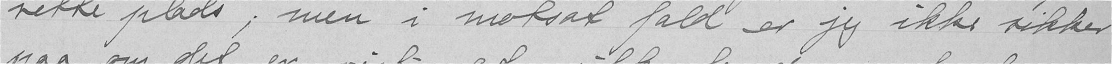rette plads, men i motsat fald er jeg ikke sikker
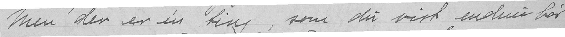Men der er en ting, som du vist endnu bør
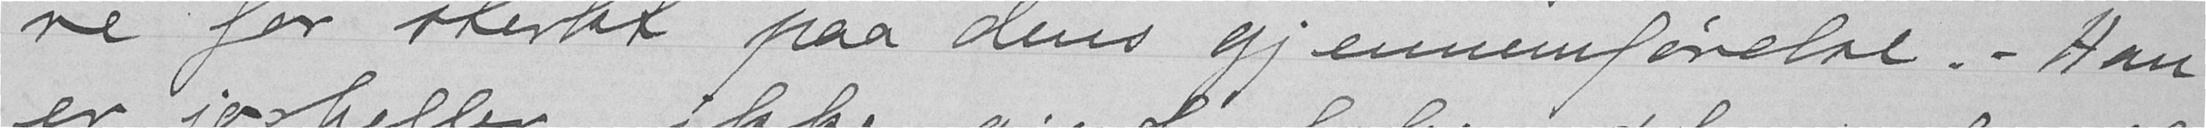re for sterkt paa dens gjennemførelse.- Han
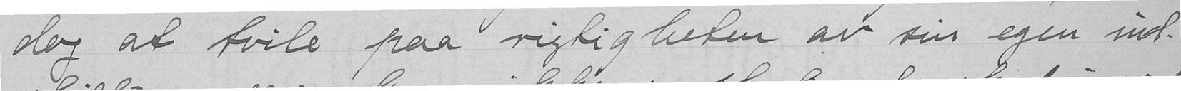dog at tvile paa rigtigheden av sin egen ind-
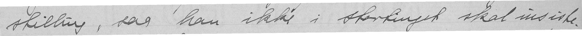stilling, saa han ikke i stortinget skal insiste-
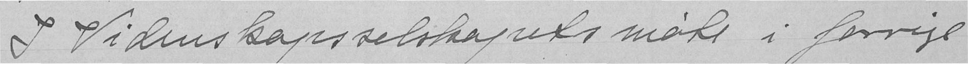I Videnskapsselskapets møte i forrige
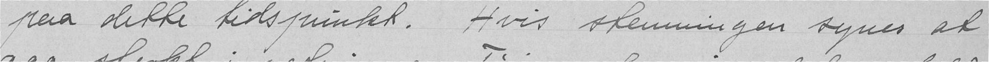paa dette tidspunkt. Hvis stemningen synes at
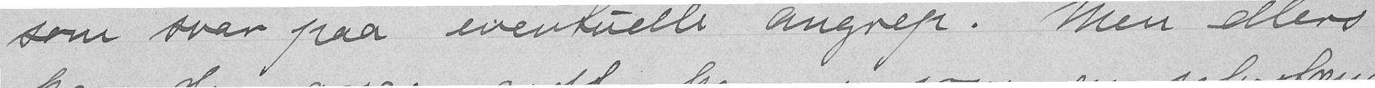som svar paa eventuelle augrep. Men ellers
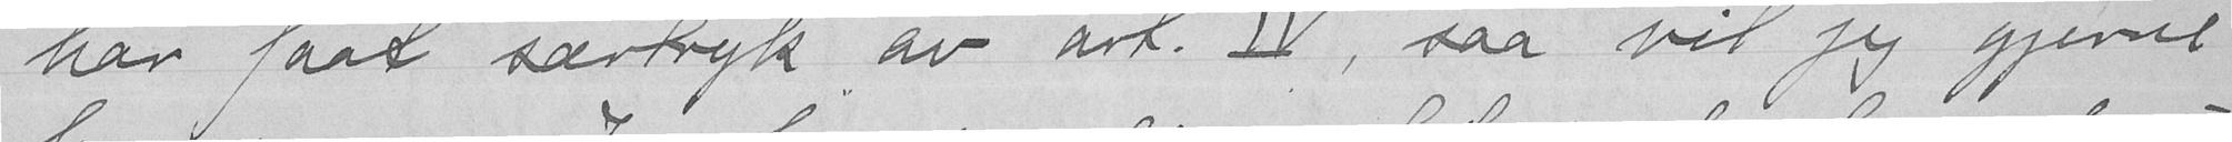har faat særtryk av art. IV, saa vil jeg gjerne
